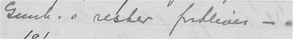Gunh.s rester forbliver - .
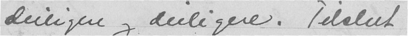deiligere og deiligere. Tilslut
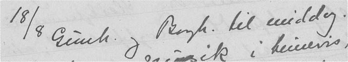18/8 Gunh. og Borgh. til middag.
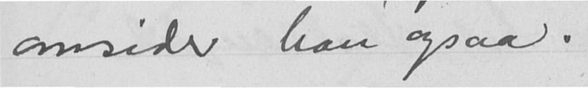omsider han ogsaa.
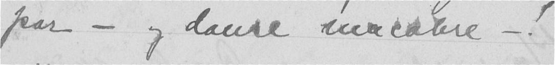par - og danse macabre - !
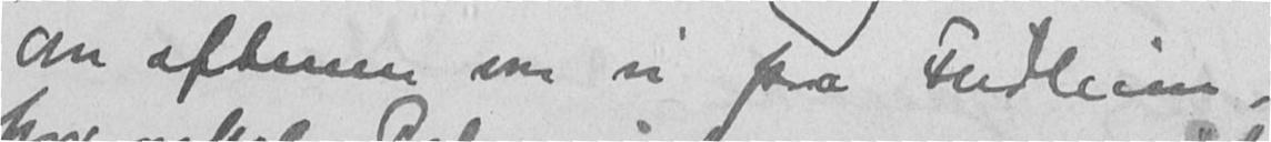Om aftenen var vi paa Fridheim,
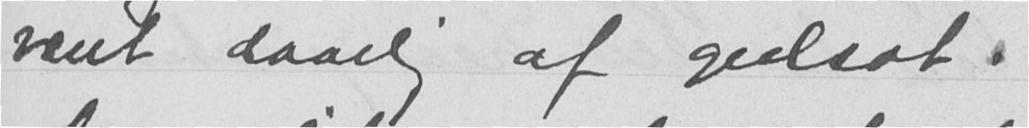rent daarlig af gulsot.
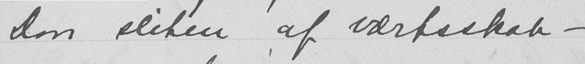Don sliten af værtsskab -
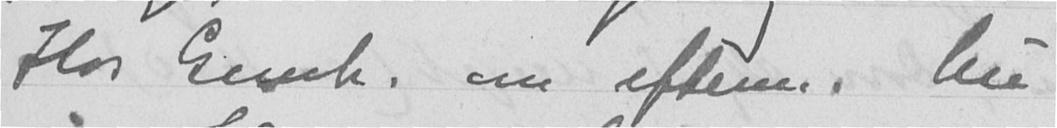Hos Gunh. om efterm. Nu
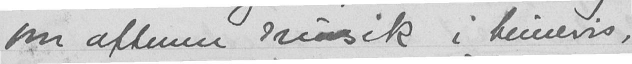Om aftenen musik i timevis,
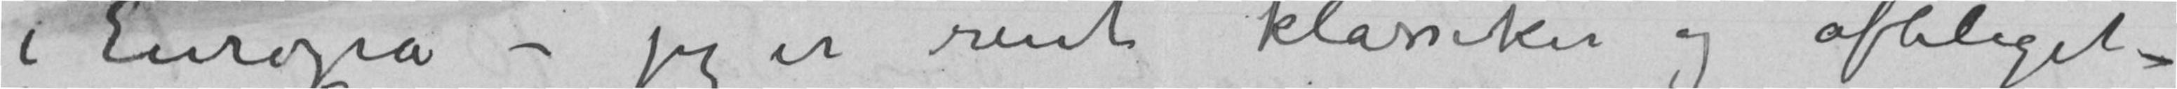i Europa – jeg er rent klassiker og afbleket –
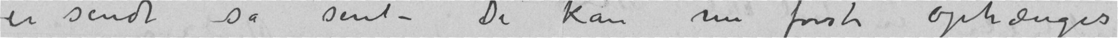er sendt så sent – De kan nu først ophænges
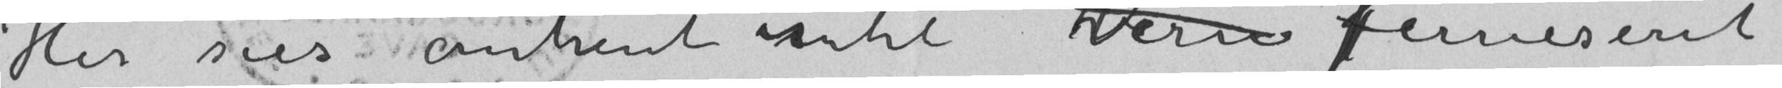Her sees omtrent intet Vvern Fferniseret
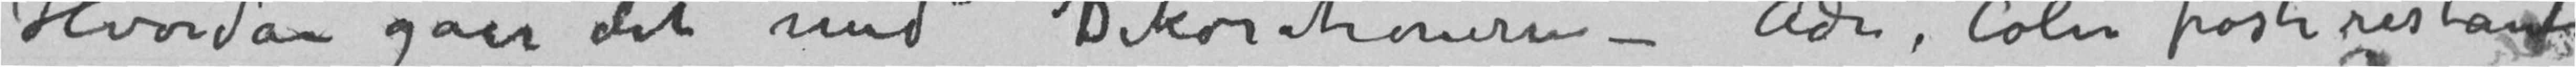Hvordan går det med Dekorationerne – Adr. Cöln poste restante
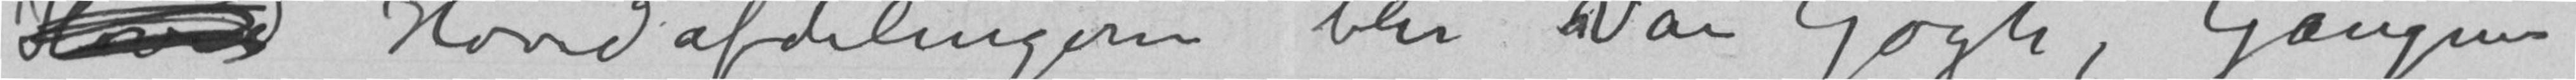Hoved Hovedafdelingerne blir Van Gogh, Gauguin
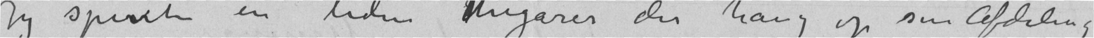Jeg spurte en liden Ungarer der hang op sin Afdeling
